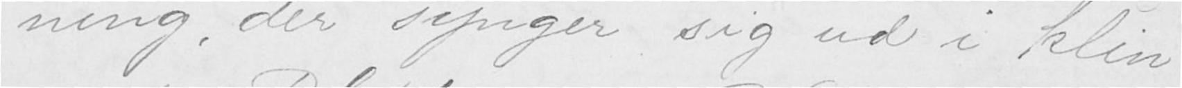ning, der synger sig ud i klin-
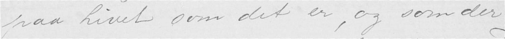paa Livet som det er, og som der
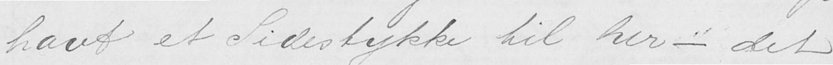havt et Sidestykke til her — det
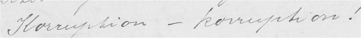Korruption — korruption!
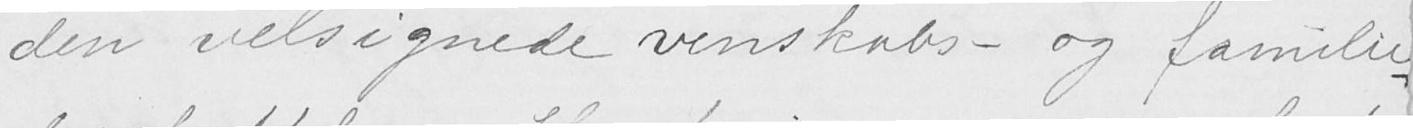den velsignede venskabs- og familie-
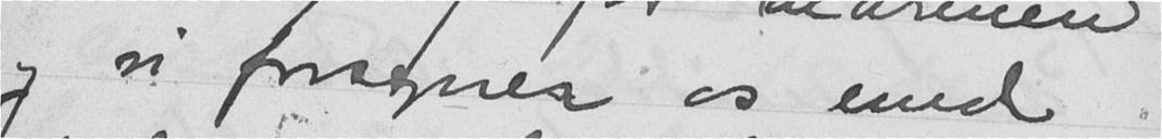og vi forsyner os med
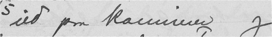ild paa kaminen og
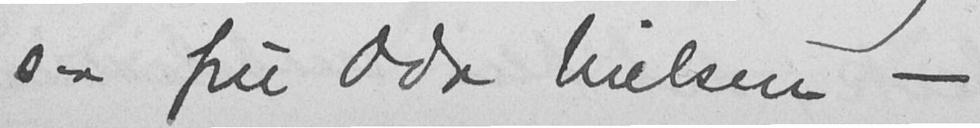saa fru Oda Nielsen -
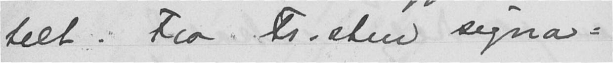telt. Fra. Fr.sten signa-
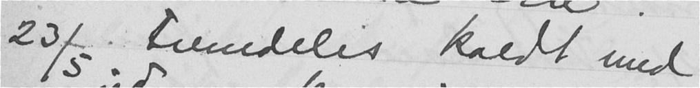23/5 Fremdeles koldt med
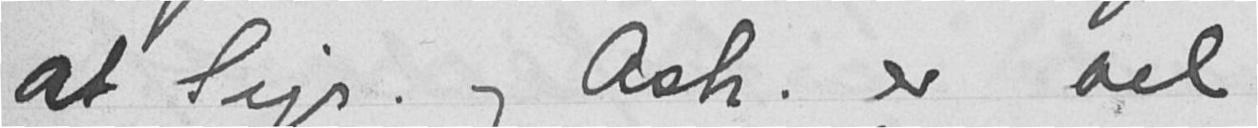at Sigr. og Astr. er vel
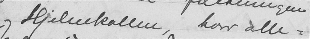og Hjelmkollen, hvor alle-
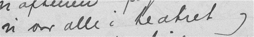vi var alle i teatret og
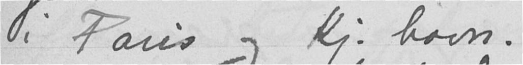i Paris og Kj. havn.
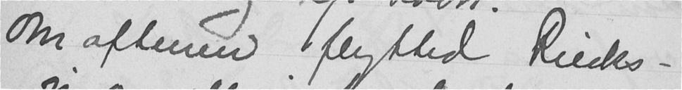Om aftenen flytted Riecks -
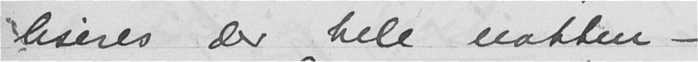liseres der hele natten -
